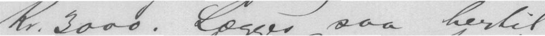Kr.3000. Lægges saa hertil
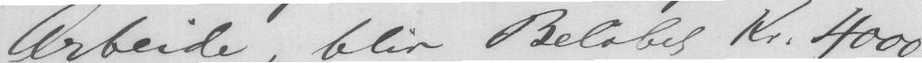Arbeide, blir Beløbet Kr.4000,
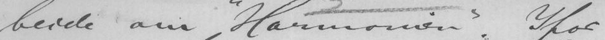beide om "Harmonien". Ifor-
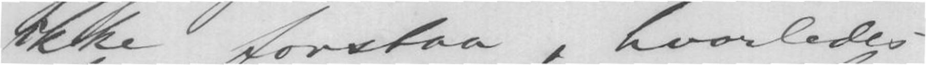ikke forstaa, hvorledes
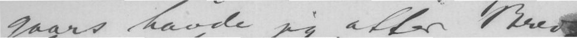gaars havde jeg atter Brev
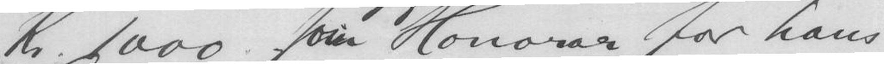Kr.1000 som Honorar for hans
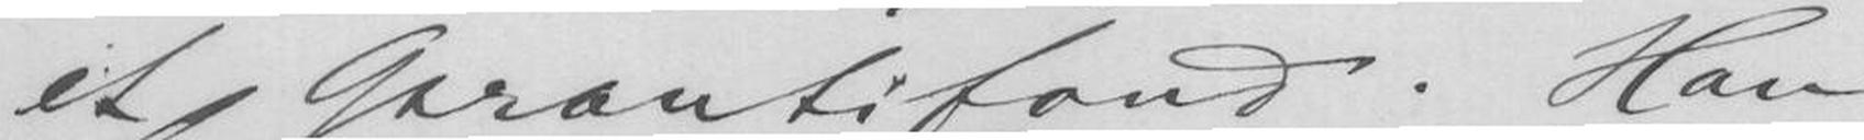et garantifond. Han
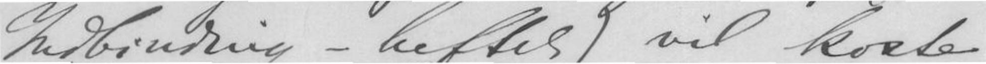(med Indbinding - heftet) vil koste
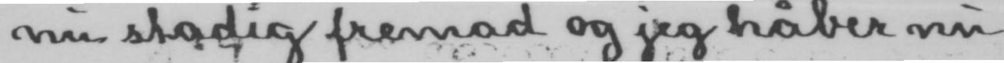nu stadig fremad og jeg håper nu
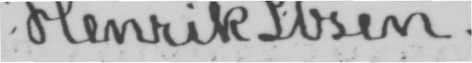Henrik Ibsen.
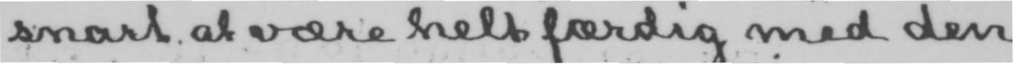snart at være helt færdig med den
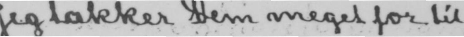Jeg takker Dem meget for til-
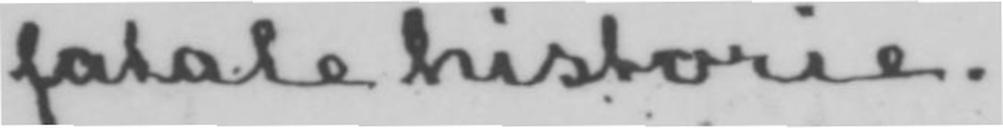fatale historie.
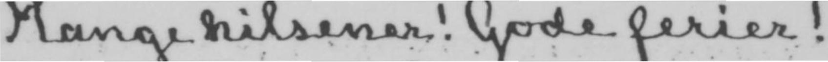Mange hilsener! Gode ferier!
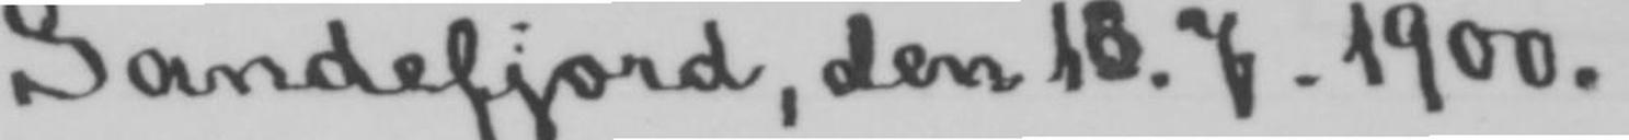Sandefjord, den 18.7.1900.
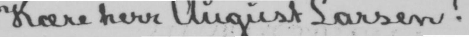Kære herr August Larsen!
